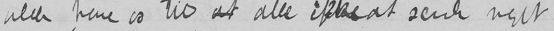vilde have os til at alle ikke at sende noget
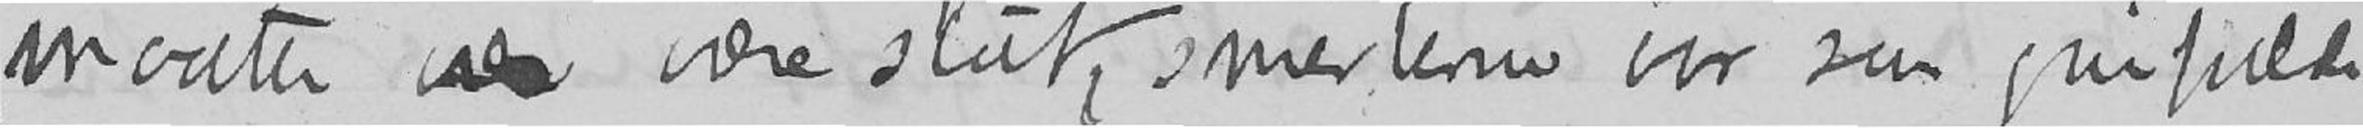maatte vær være slut, smerterne var saa grufulde.
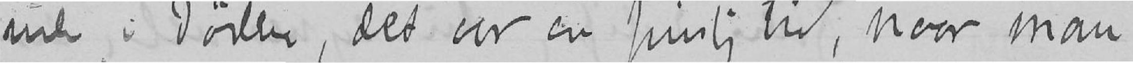inde i døden, det var en pinlig tid, naar man
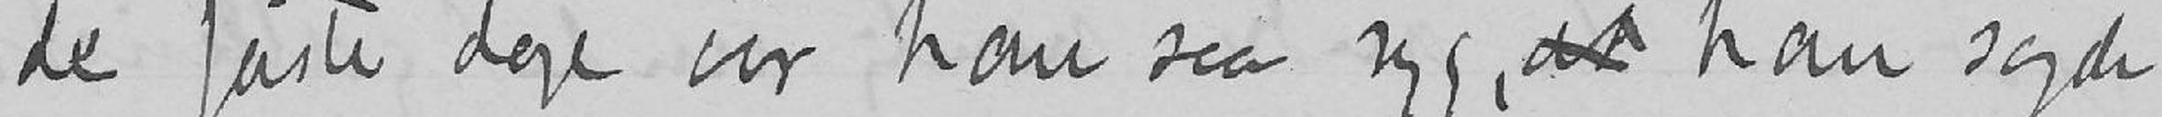de første dage var han saa syg, at han sagde
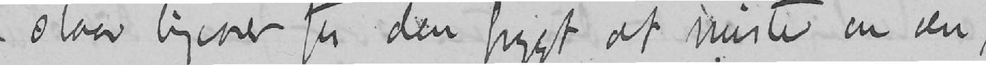staar ligeover for den frygt at miste en ven,
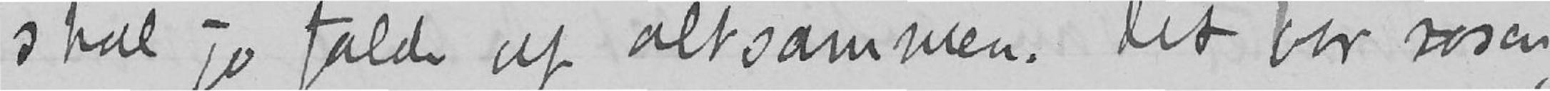skal jo falde af altsammen. Det var rosen,
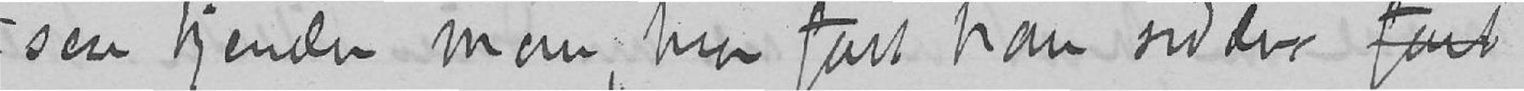saa kjender man, hvor fast han sidder fast
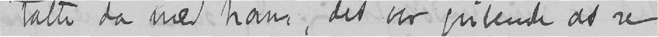talte da med ham, det var gribende at se
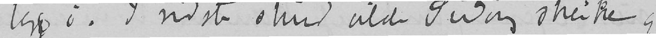tag i. I sidste stund vilde Sinding streike og
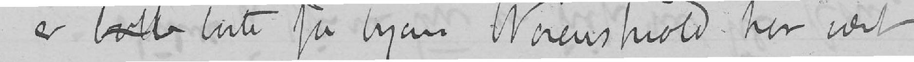er borte fra byen Werenskiold har vært
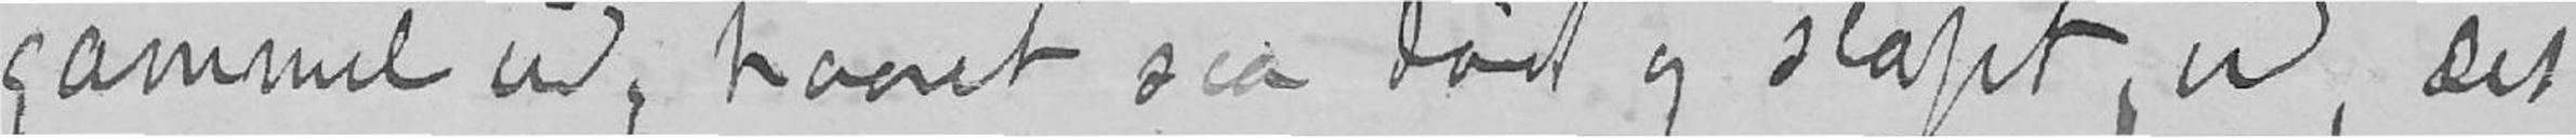gammel ud, haaret saa dødt og slapt ud, det
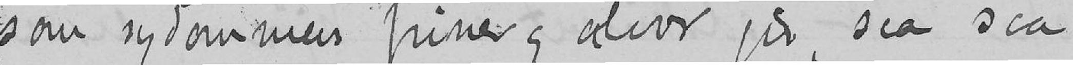som sydommers piner og alvor gir, saa saa
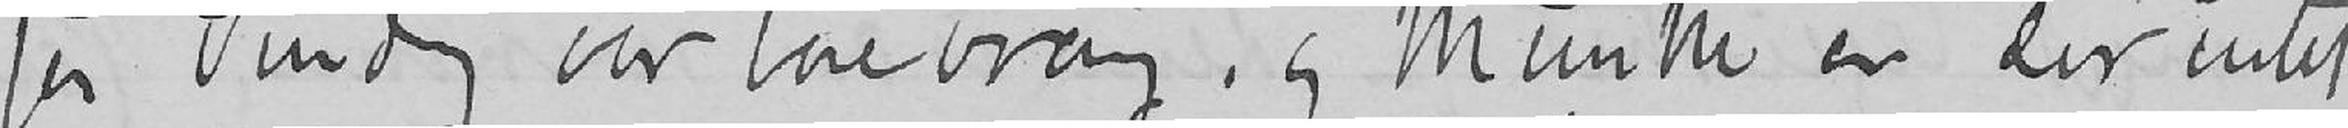for Sinding var bare vrang, og Munthe er der intet
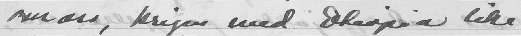over oss, krigen med Etiopia like
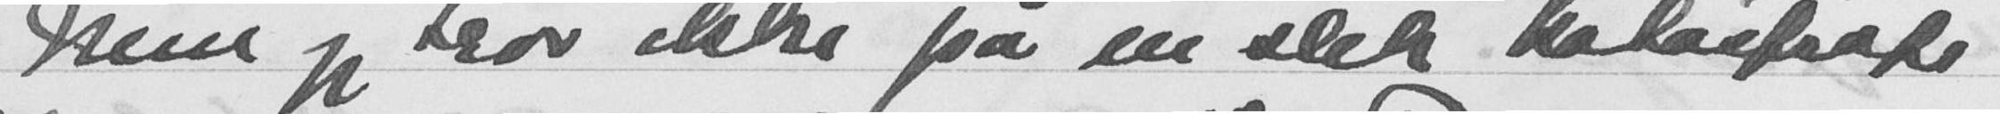Men jeg tror ikke på en slik katastrofe
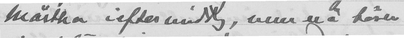Märtha i eftermiddag, men nå hører
